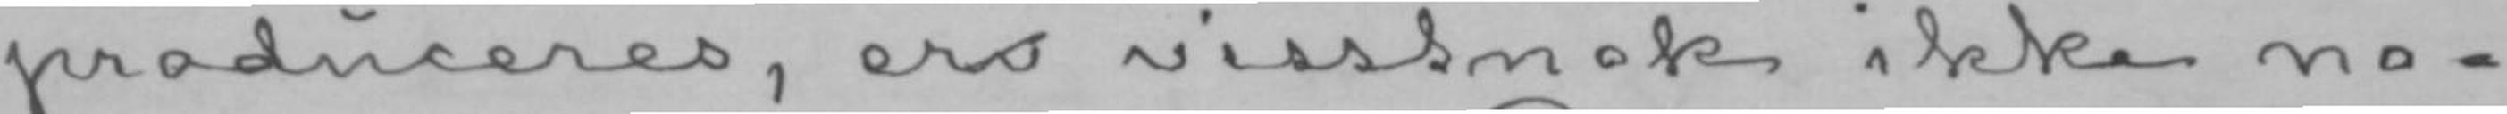produceres, er visstnok ikke no-
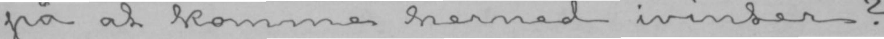på at komme herned ivinter?
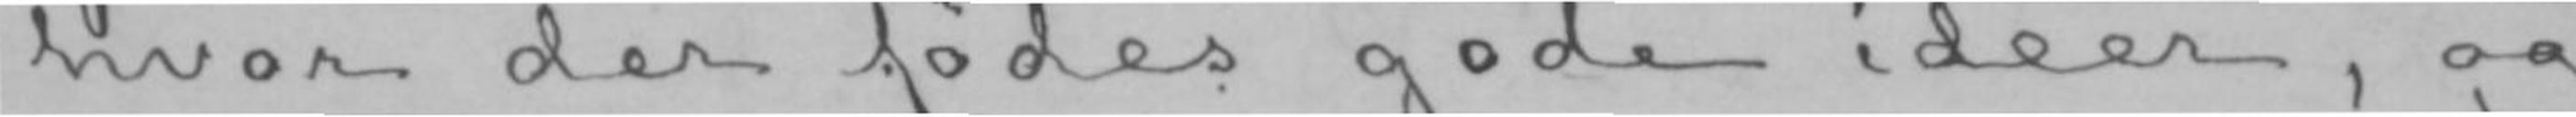hvor der fødes gode ideer, og
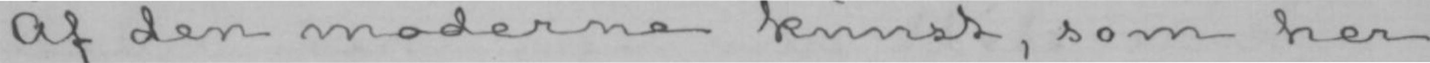Af den moderne kunst, som her
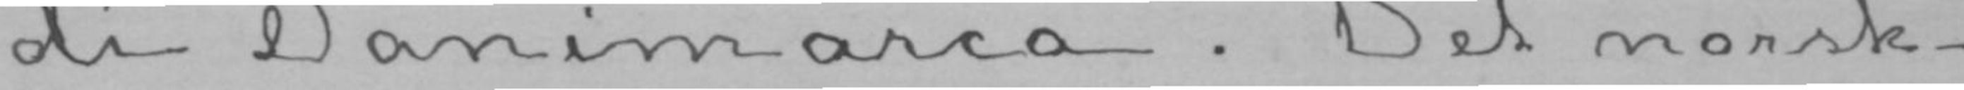di Danimarca. Det norsk-
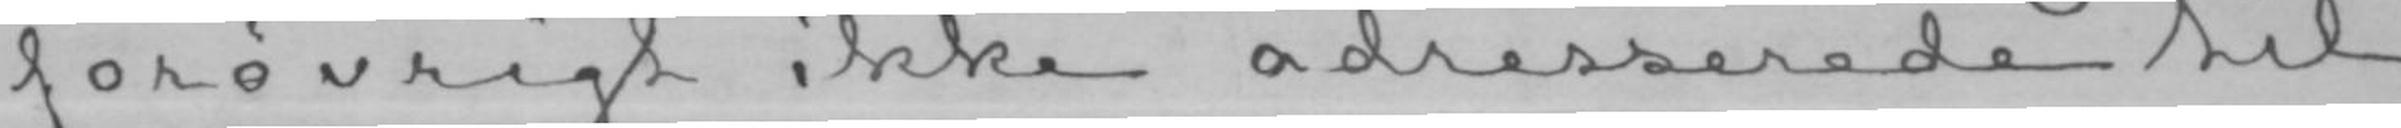forøvrigt ikke adresserede til
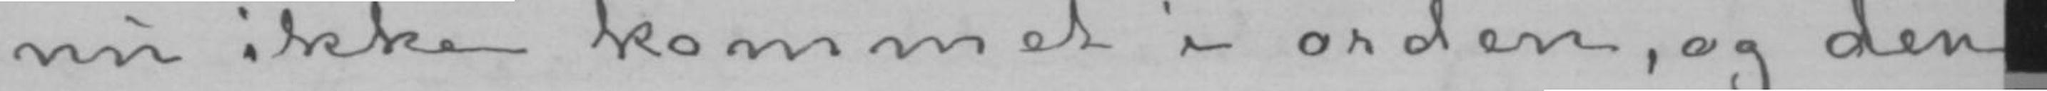nu ikke kommet i orden, og den
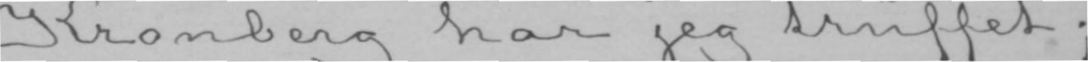Kronberg har jeg truffet;
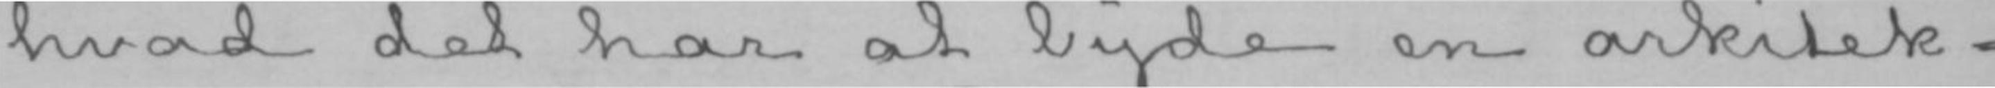hvad det har at byde en arkitek-
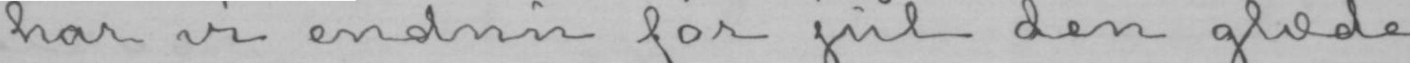har vi endnu før jul den glæde
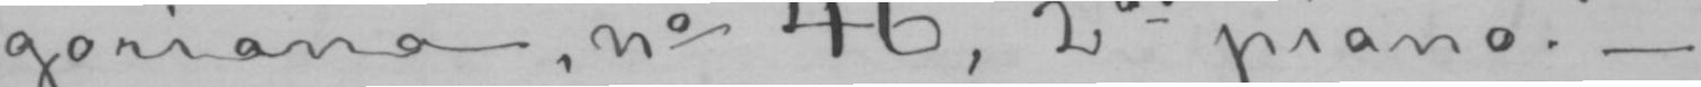goriana, no 46, 2d. piano.-
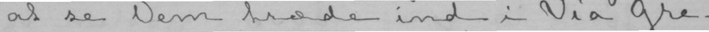at se Dem træde ind i Via Gre-
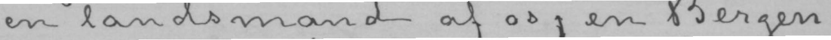en landsmand af os;, en Bergen-
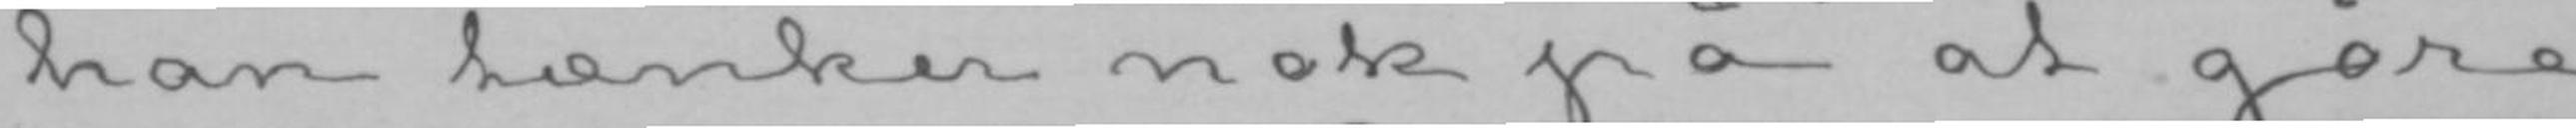han tænker nok på at gøre
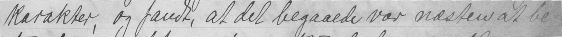karakter, og fandt, at det begaaede var næsten at be-
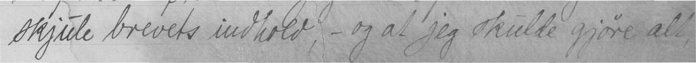skjule brevets indhold, - og at jeg skulde gjøre alt,
